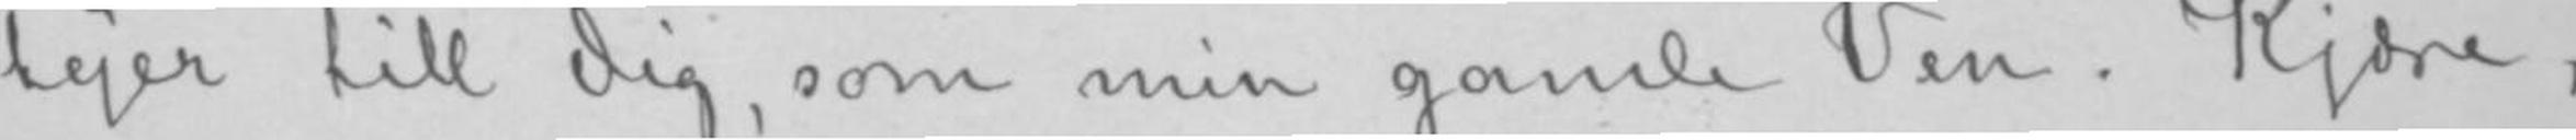tyer till Dig, som min gamle Ven. Kjære,
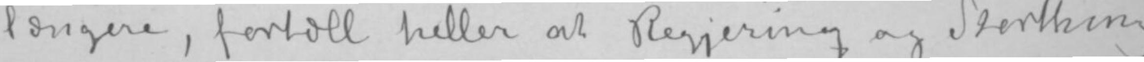længere, fortæll heller at Regjering og Storthing
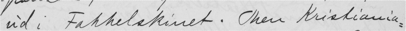ud i Fakkelskinet. Men Kristiania-
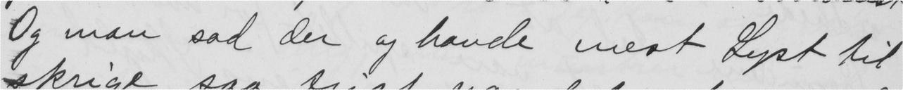Og man sad der og havde mest Lyst til
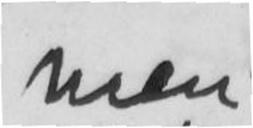men
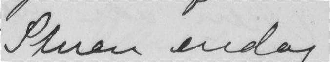Stuen endog
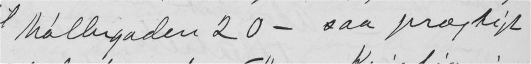Møllergaden 20 - saa prægtigt
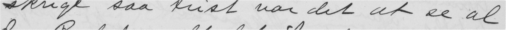skrige saa trist var det at se al
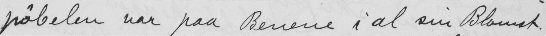pøbelen var paa Benene i al sin Blomst.
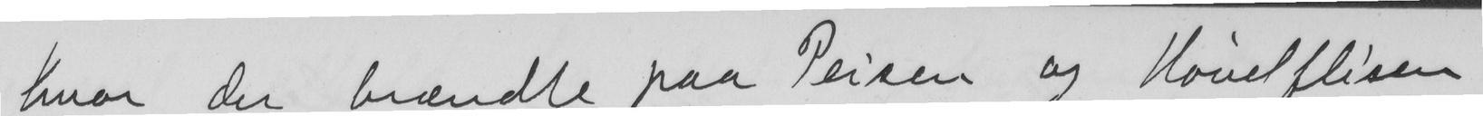hvor der brændte paa Peisen og Høvelflisen
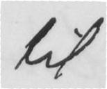til
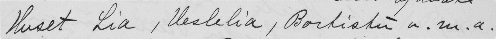Huset Lia, Veslelia, Bortistu v.m.a.
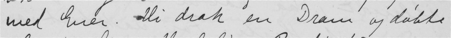med Ener. Vi drak en Dram og døbte
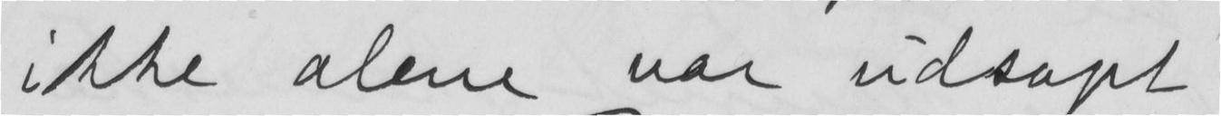ikke alene var udsopt
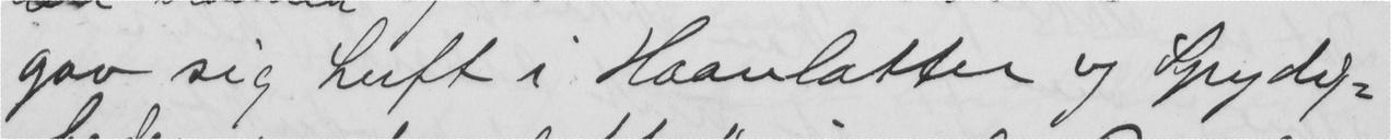gav sig Luft i Haanlatter og Spydig-
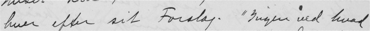hver efter sit Forslag. "Ingen ved hvad
